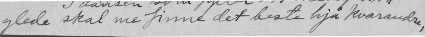glede skal me finne det beste hjå kvarandre,
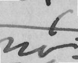no
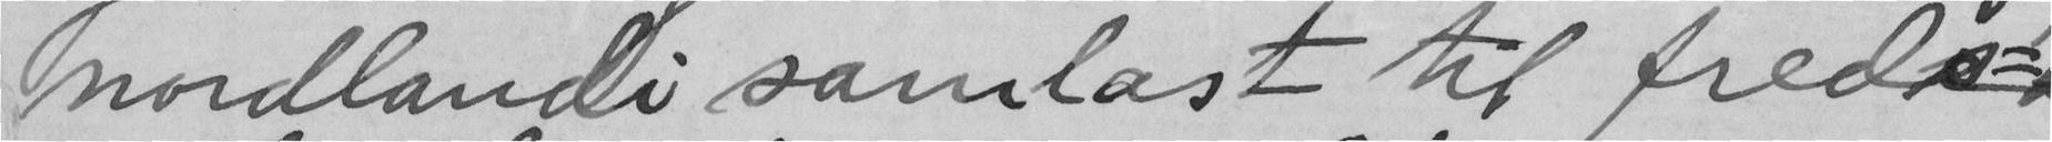nordlandi samlast til freds-
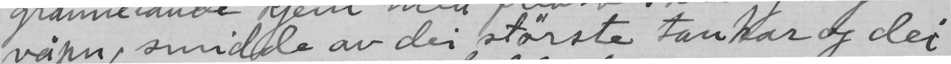våpen, smidde av dei største tankar og dei
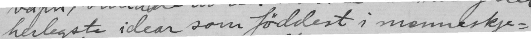herlegste idear som føddest i menneskje-
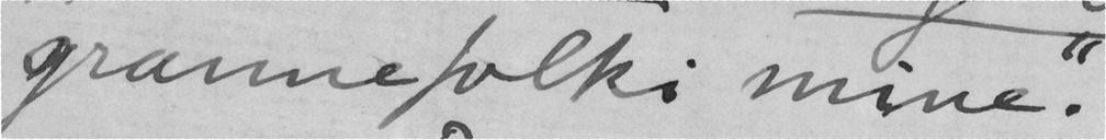grannefolki mine."
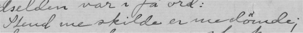Stend me skilde er me dømde;
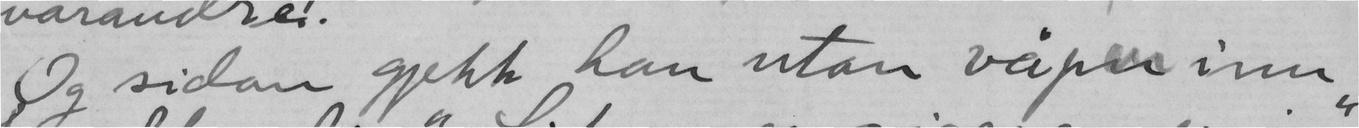Og sidan gjekk han utan våpen inn
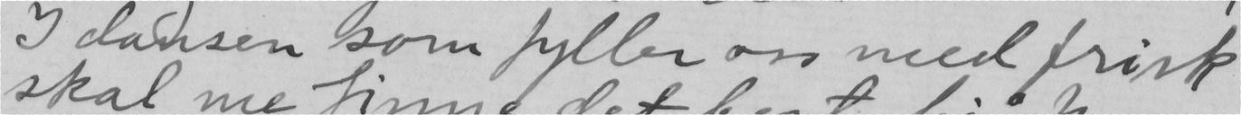I dansen som fyller oss med frisk
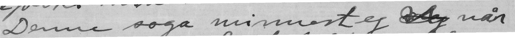Denne soga minnest eg og når
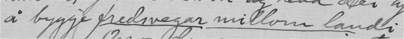å byggje fredsvegar millom landi.
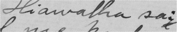Hiawatha sa:
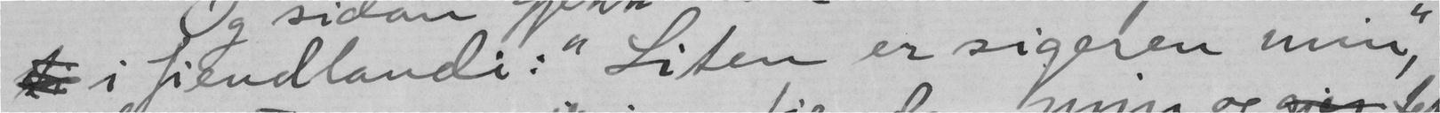i fiendlandi: "Liten er sigeren min",
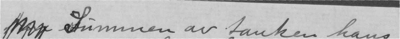Summen av tanken hans
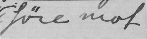føre mot
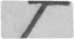7
